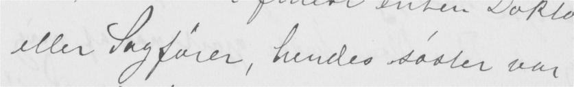eller Sagfører, hendes søster var
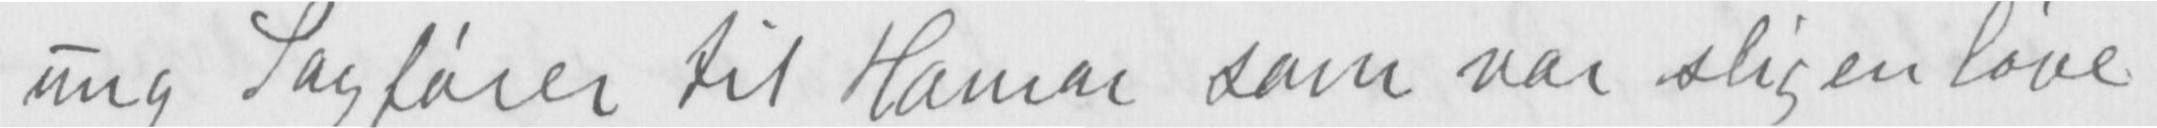ung Sagfører til Hamar som var slig en løve
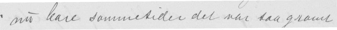nu bare sommetider det var saa gromt
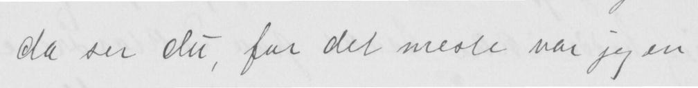da ser du, for det meste var jeg en
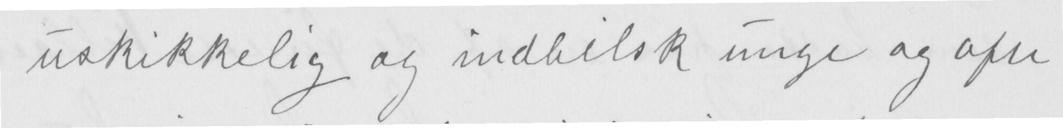uskikkelig og indbilsk unge og ofte
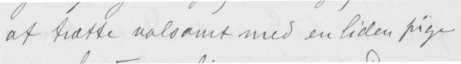at trætte volsomt med en liden pige
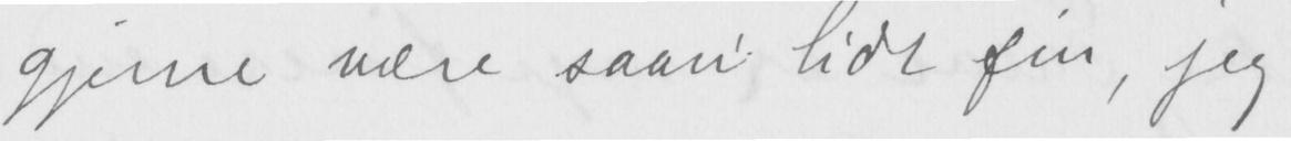gjerne være saan lidt fin, jeg
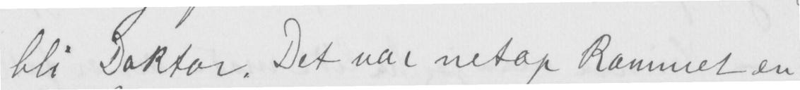bli Doktor. Det var netop kommet en
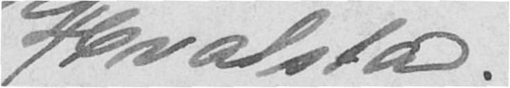Hvalstad.
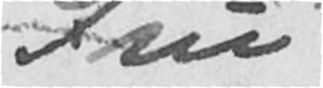Frue
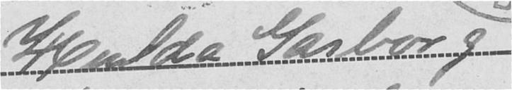Hulda Garborg
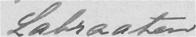Labraaten
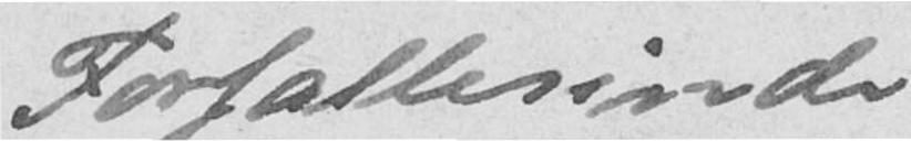Forfatterinde
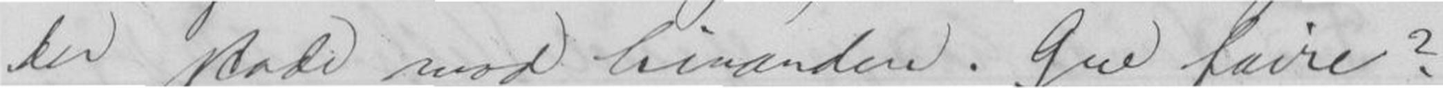der skæde mod hinanden. Que faire?
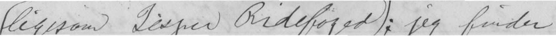(ligesom Jesper Ridefoged): jeg finder
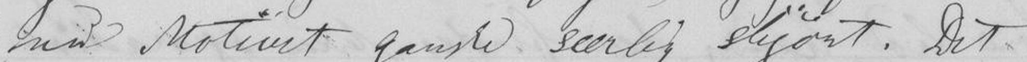nu Motivet ganske særlig skjønt. Det
